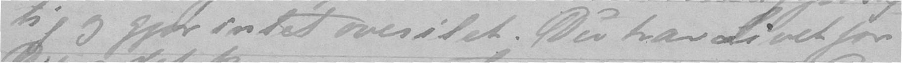tig og gjør intet overilet. Du har Livet for
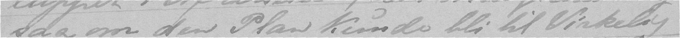saa, om den Plan kunde bli til Virkelig-
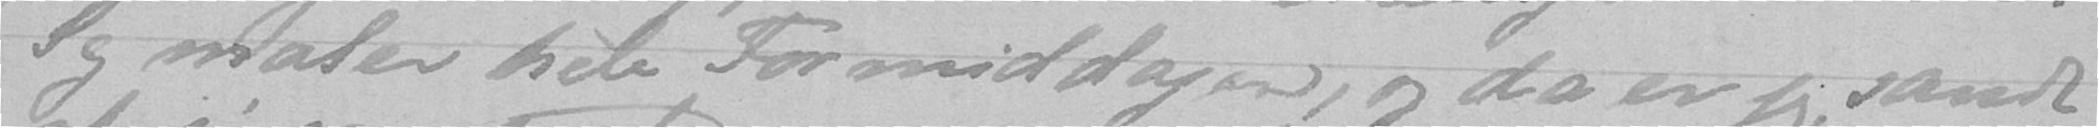Jeg maler hele Formiddagen, og da er jeg, sandt
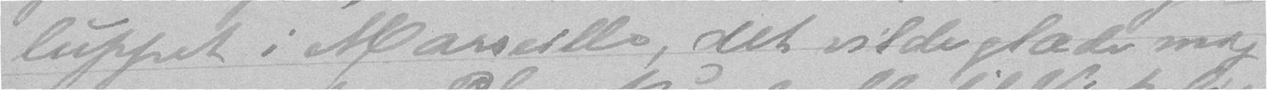luppet i Marseille, det vilde glæde mig
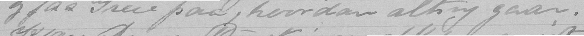og faa Greie paa, hvordan alting gaar.
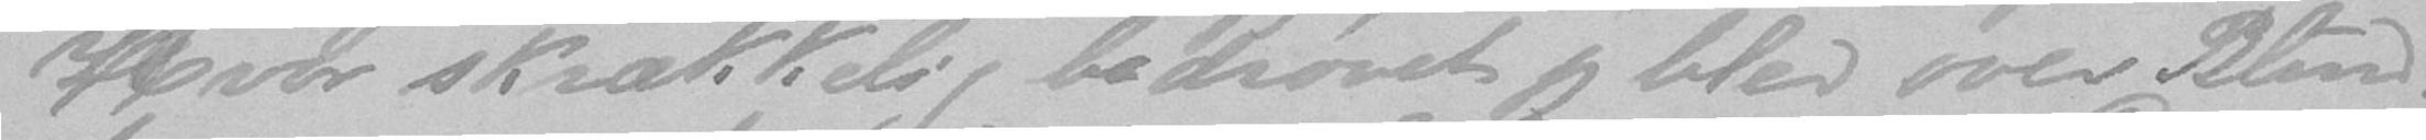Hvor skrækkelig bedrøvet jeg blev over Blind-
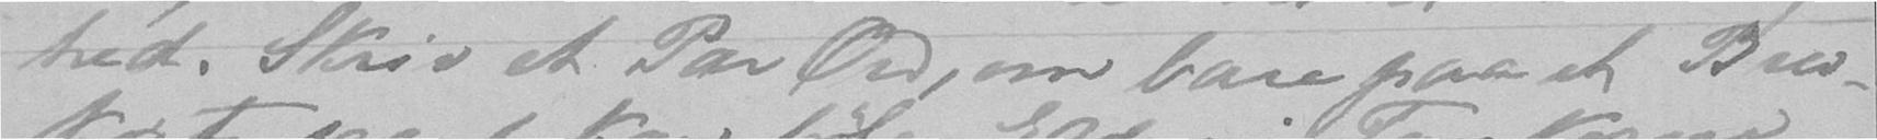hed. Skriv et Par Ord, om bare paa et Brev-
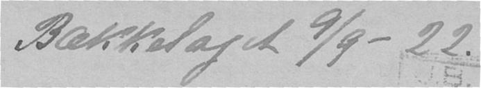Bækkelaget 9/9-22.
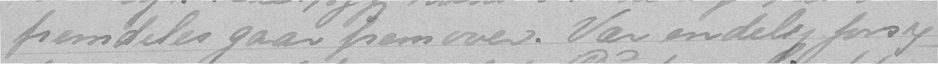fremdeles gaar fremover. Vær endelig forsig-
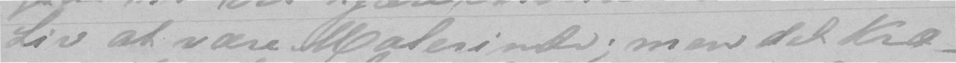Liv at være Malerinde; men det kræ-
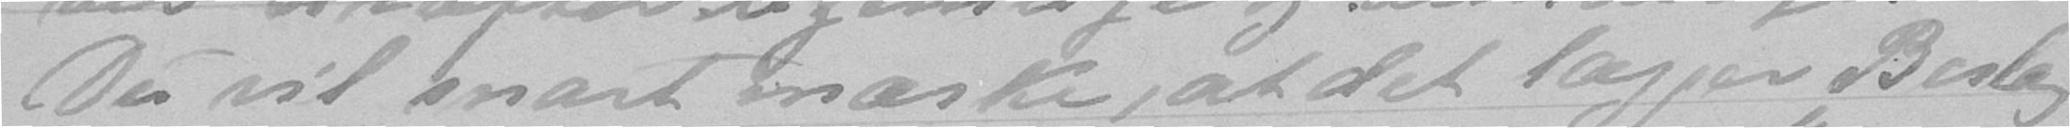Du vil snart mærke, at det lægger Beslag
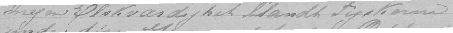megen Elskværdighet blandt Tyskerne
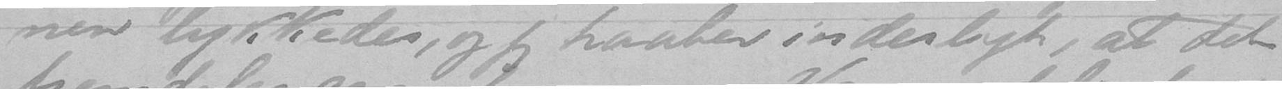nen lykkedes, og jeg haaber inderligt, at det
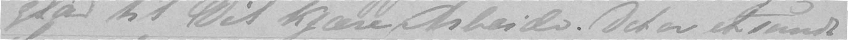glad til Dit kjære Arbeide. Det er et sundt
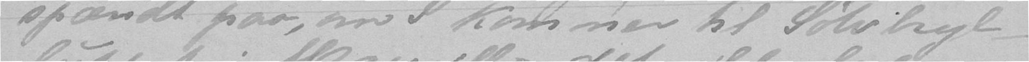spændt paa, om I kommer til Sølvbryl-
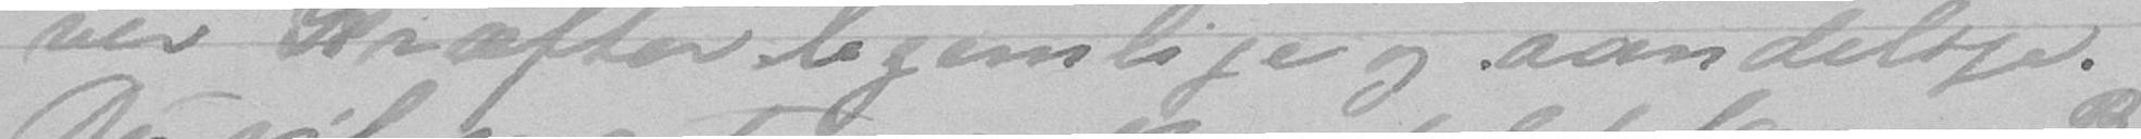ver Kræfter legemlige og aandelige.
